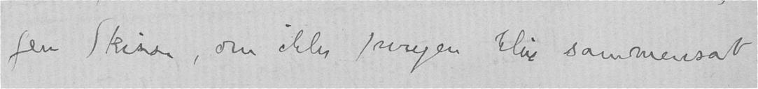gen Skisser, om ikke Juryen blive sammensat
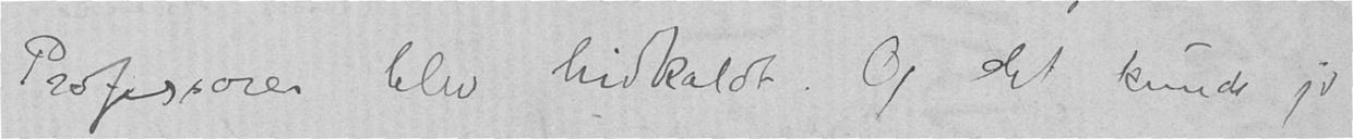Professorer blev hidkaldt. Og det kunde jo
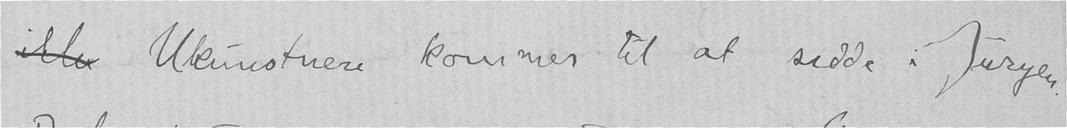Ukunstnere kommer til at sidde i Juryen.
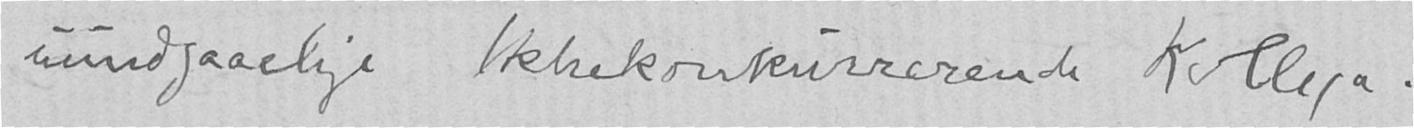uundgaaelige Ikkekonkurrerende Kollega.
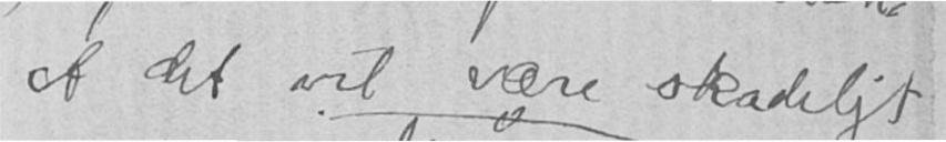at det vil være skadeligt
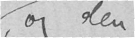og den
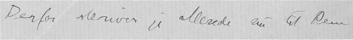Derfor skriver jeg allerede nu til Dem
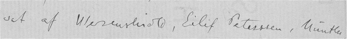sat af Werenskiold, Eilif Peterssen, Munthe,
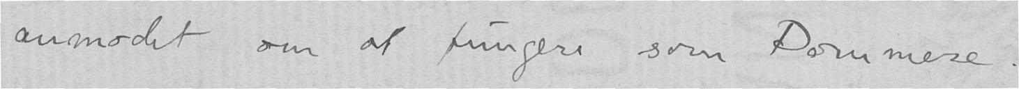anmodet om af fungere som Dommere.
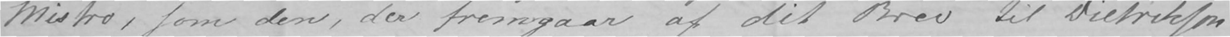Mistro, som den, der fremgaar af dit Brev til Dietrikson.
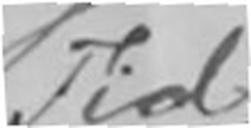Tid
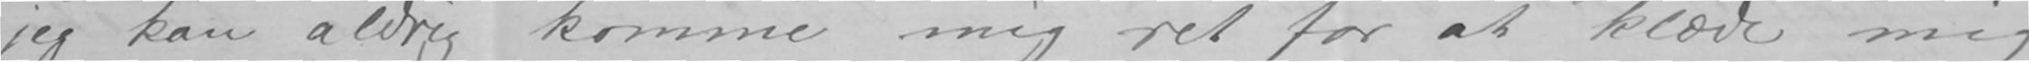jeg kan aldrig komme mig ret for at klæde mig
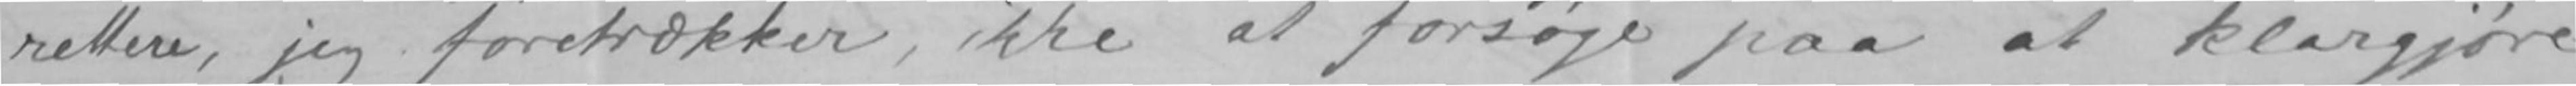rettere, jeg foretrækker, ikke at forsøge paa at klargjøre
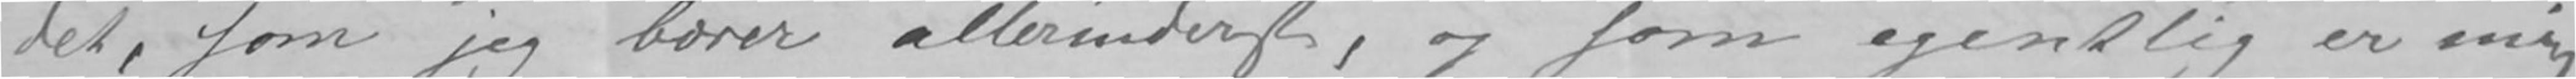det, som jeg bærer allerinderst, og som egentlig er mig
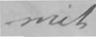mit
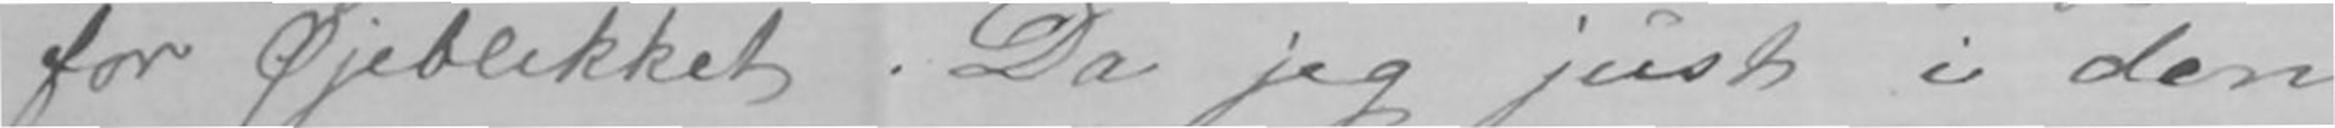for Øjeblikket. Da jeg just i den
